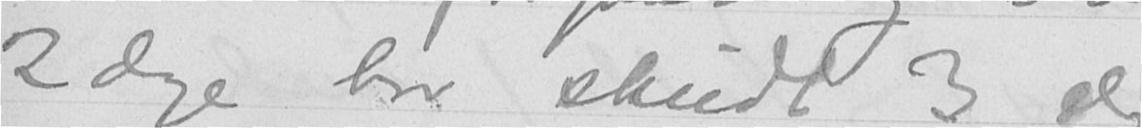2 dage har skudt 3 elg
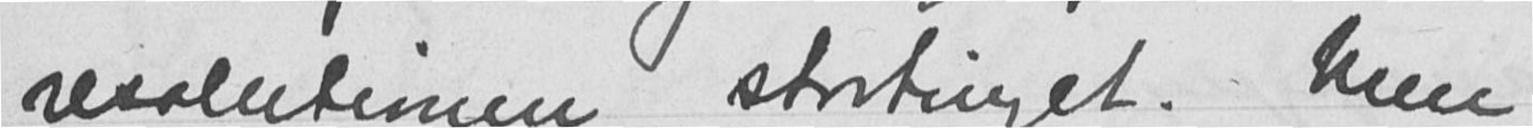resolutionen stortinget. Men
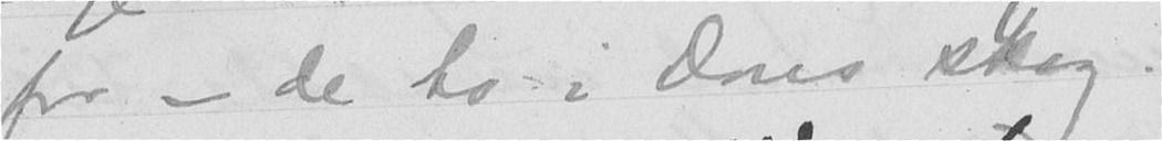for - de to i Dons skog.
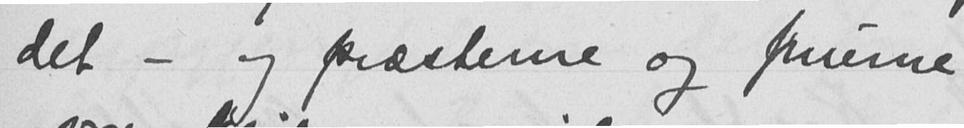det - og præsterne og fruerne
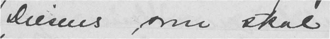Diesens, som skal
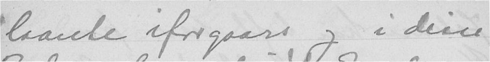laante iforgaars og i disse
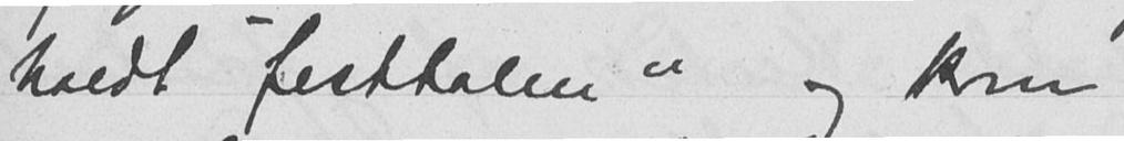holdt "festtalen" og kom
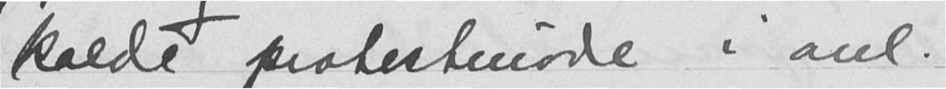kalde protestmøde i anl.
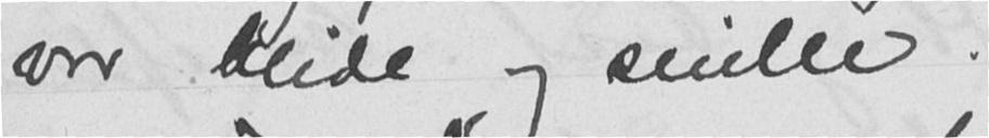var blide og snille.
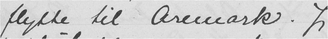flytte til Aremark. Jeg
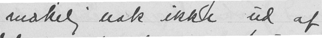mærkelig nok ikke ud af
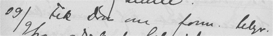29/9 Fik Don om form. telgr.
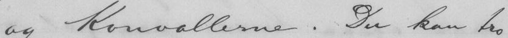og Konvallerne. Du kan tro,
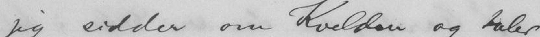jeg sidder om Kvelden og taler
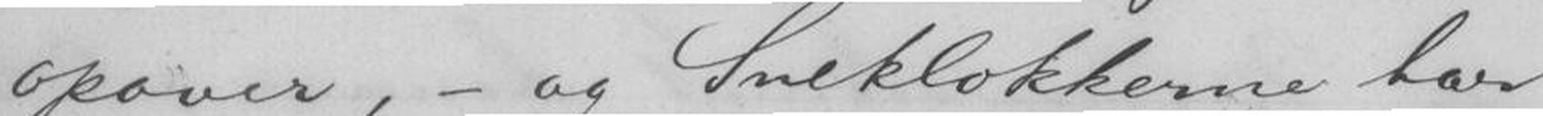opover, - og Sneklokkerne har
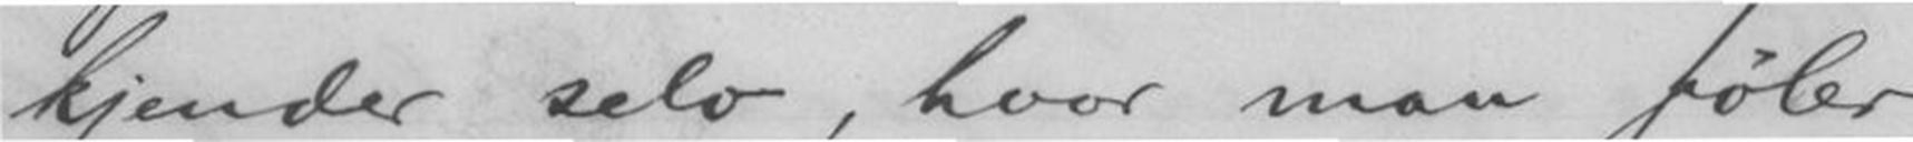kjender selv, hvor man føler
